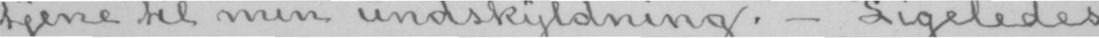tjene til min undskyldning.- Ligeledes
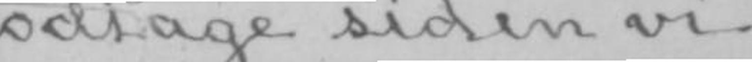odtage siden vi
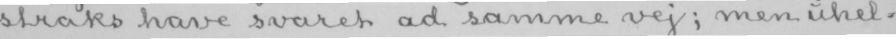straks have svaret ad samme vej; men uhel-
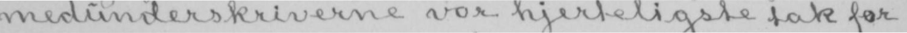medunderskriverne vor hjerteligste tak for
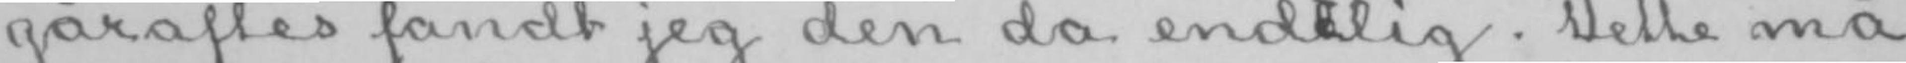gåraftes fandt jeg den da endlelig. Dette må
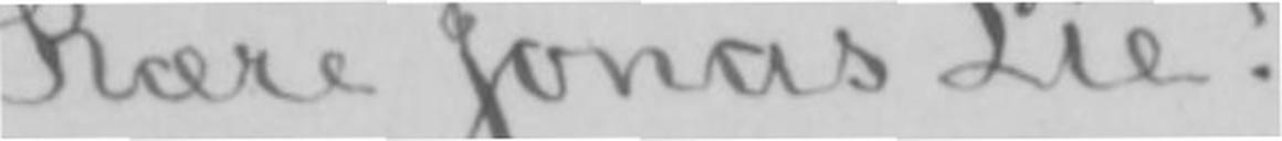Kære Jonas Lie!
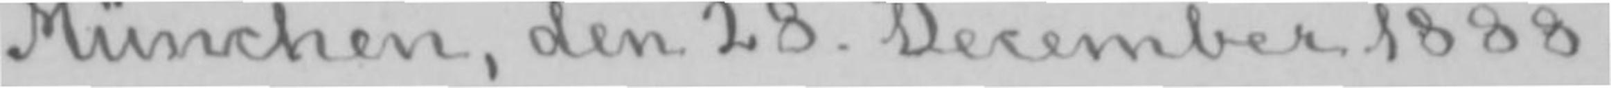München, den 28. December 1888.
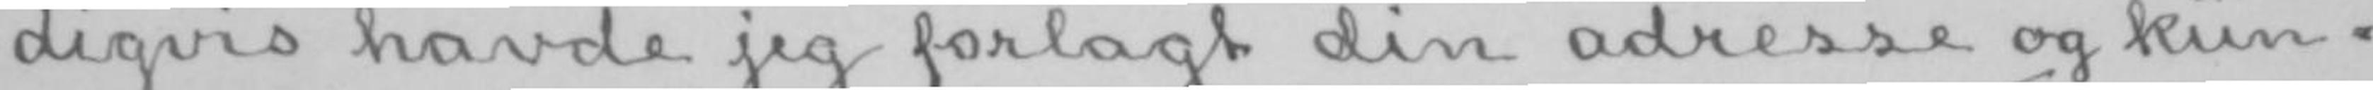digvis havde jeg forlagt din adresse og kun-
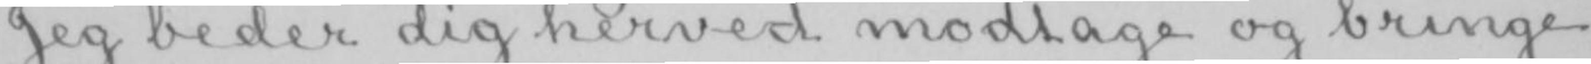Jeg beder dig herved modtage og bringe
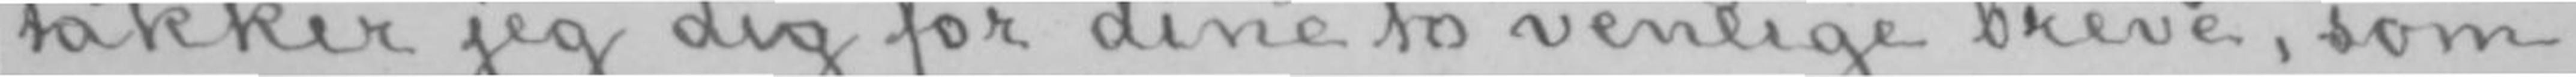takker jeg dig for dine to venlige breve, som
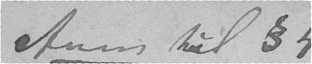Anm til § 4
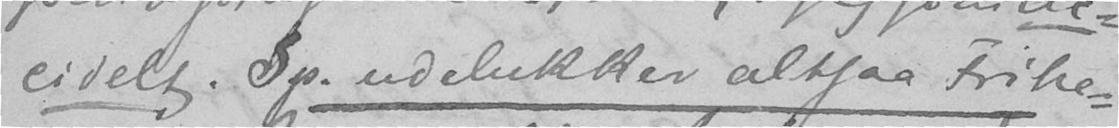cidelt. Sp. udelukker altsaa Frihe-
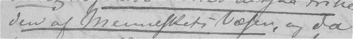den af Menneskets Væsen, og da
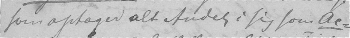som optager alt Andet i sig som Ac-
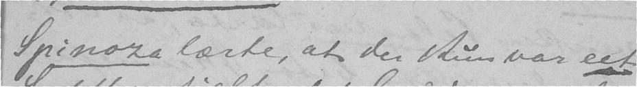Spinoza lærte, at der kun var eet
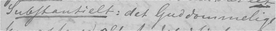Substantielt: det Guddommelige
